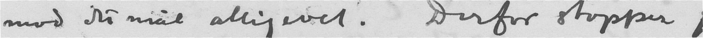mod det mål alligevel. Derfor stopper jeg
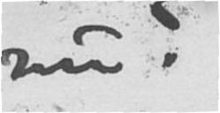nu?
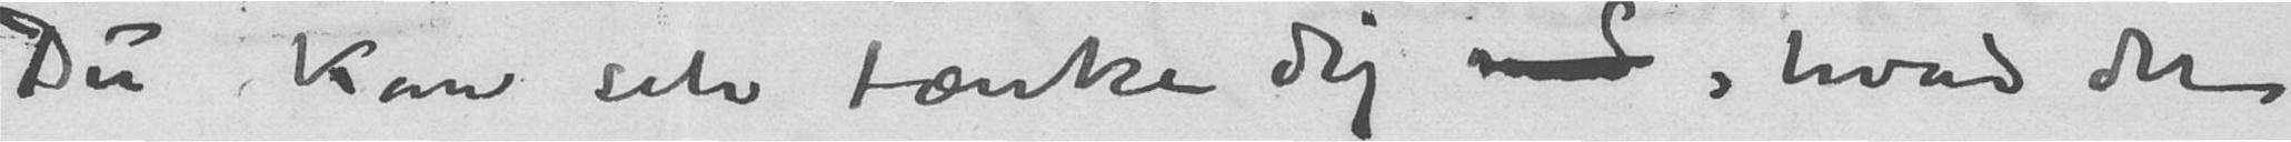Du kan selv tænke dig med, hvad der
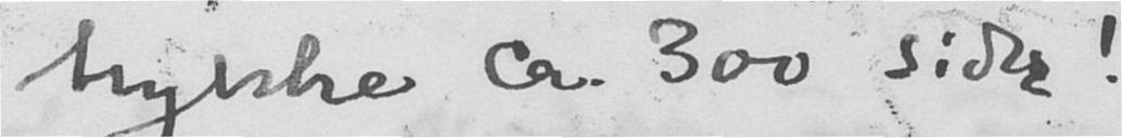trykke ca. 300 sider!
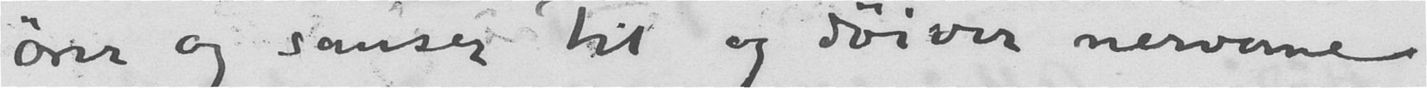ører og sanser til og døiver nerverne
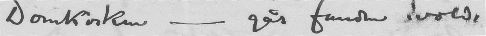Domkirken - går fanden ivold.
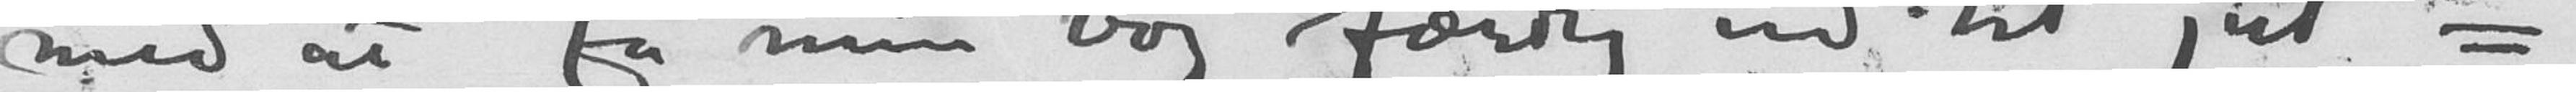med at få min bog færdig ud til jul =
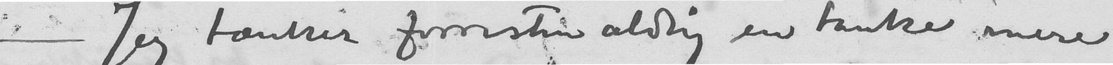- Jeg tænker forresten aldrig en tanke mere
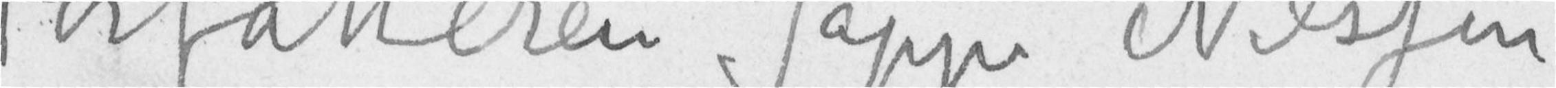Forfatteren Jappe Nilssen
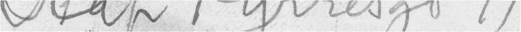Olaf Kyrres gd 11
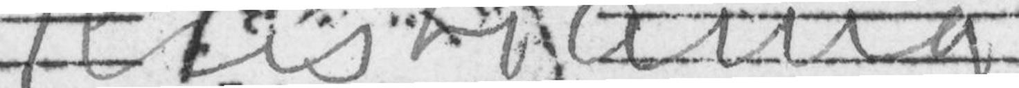Kristiania
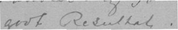godt Resultat.
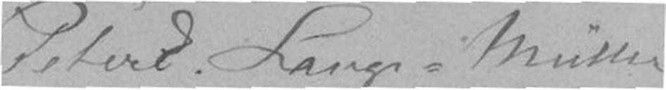Peter Lange Muller
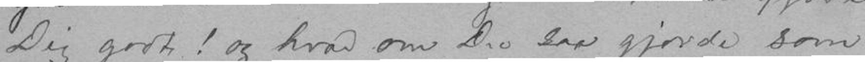Dig godt! og hvad om Du saa gjorde som
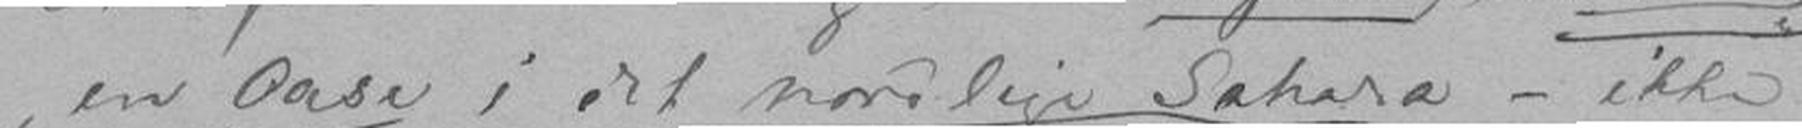, en Oase i det nordlige Sahara - ikke
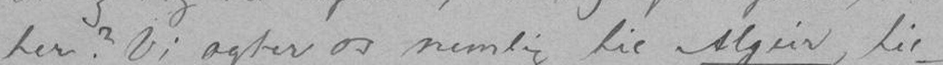ter? Vi agter os nemlig til Algier til
